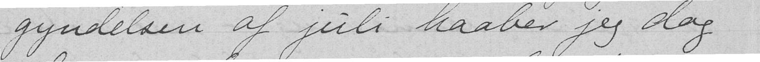gyndelsen af juli haaber jeg dog
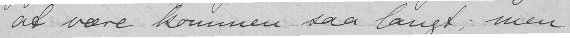at være kommen saa langt; men
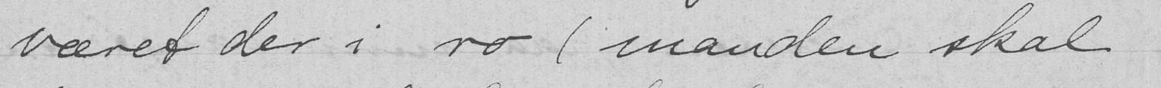været der i ro (manden skal
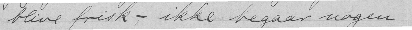blive frisk - ikke begaar nogen
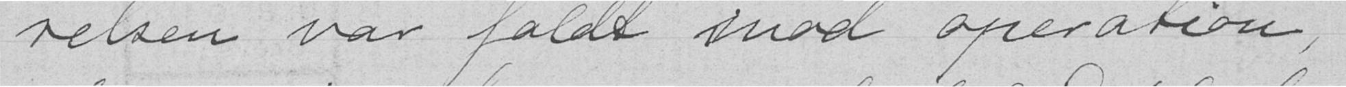relsen var faldt mod operation,
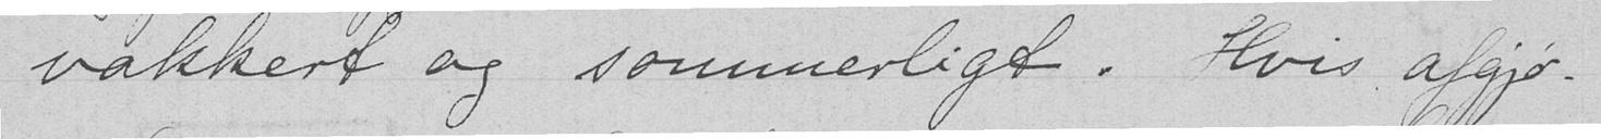vakkert og sommerligt. Hvis afgjø-
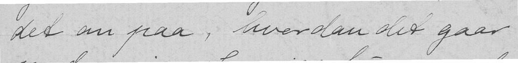det an paa, hvordan det gaar
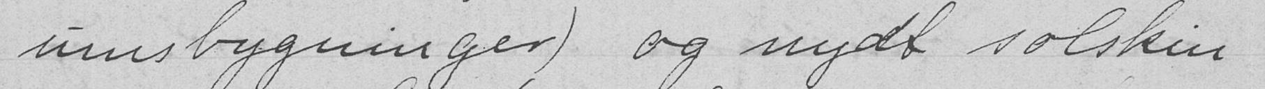umsbygninger) og nydt solskin
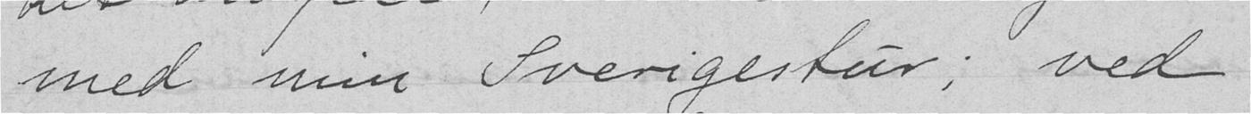med min Sverigestur; ved
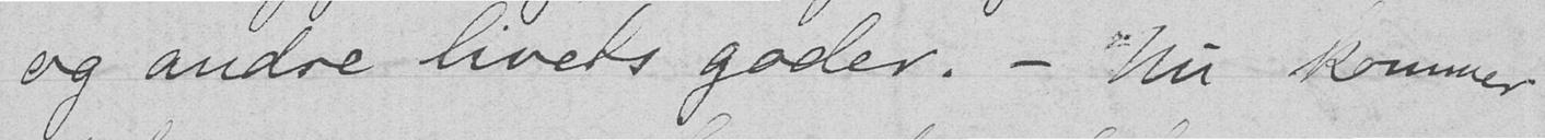og andre livets goder. - Nu kommer
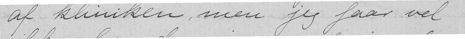af kliniken, men jeg faar vel
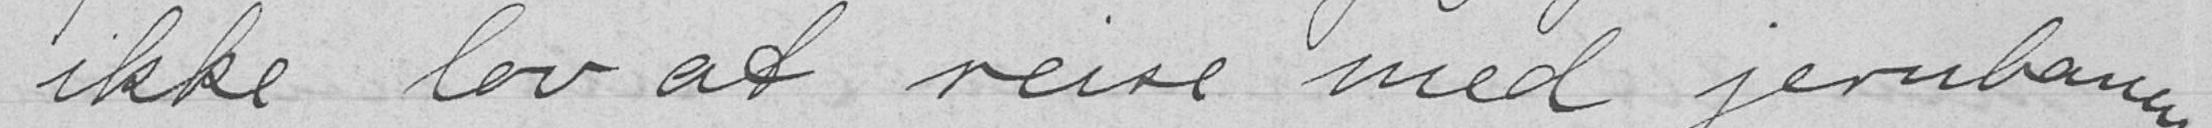ikke lov at reise med jernbanen
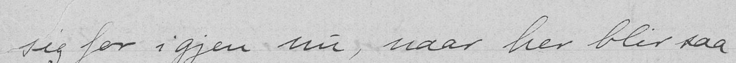sig for igjen nu, naar her blir saa
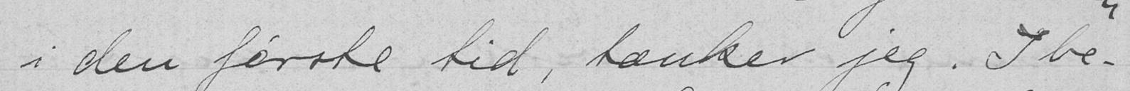i den første tid, tænker jeg. I be-
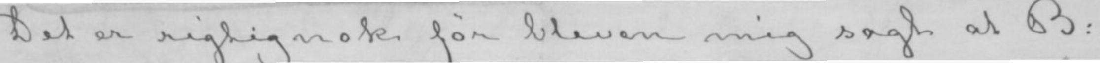Det er rigtignok før bleven mig sagt at B:
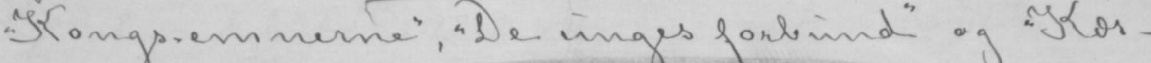«Kongs-emnerne», «De unges forbund» og «Kær-
